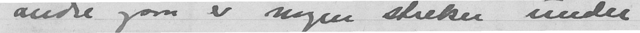andre ogsaa er nogen streker under
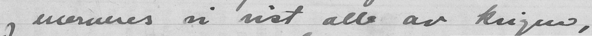enerveres vi vist alle av krigen,
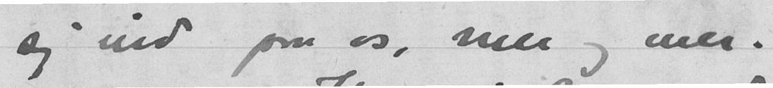sig ind paa os, mer og mer.
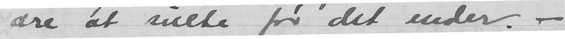ære at sulte før det ender. -
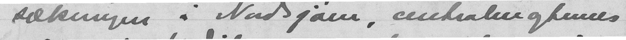sækningen i Nordsjøen, centralmagtenes
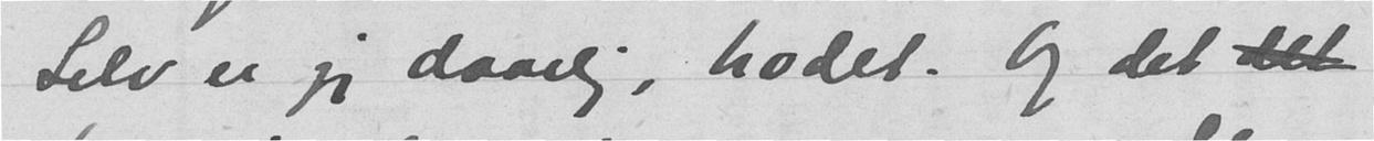Selv er jeg daarlig i hodet. Og det det
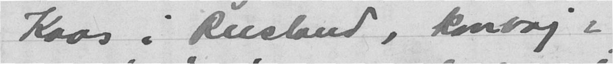Kaos i Rusland, konvoj i
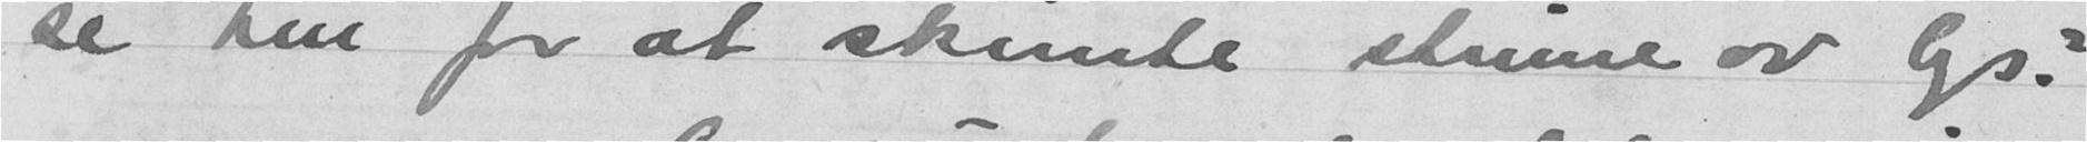se hen for at skimte strime av lys?
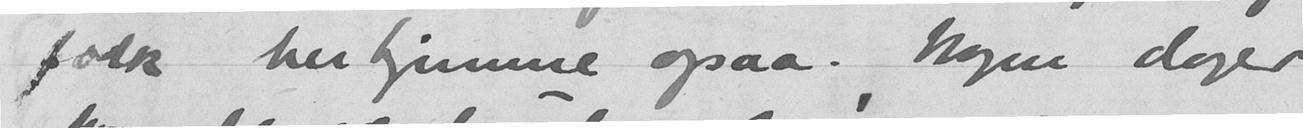folk her hjemme ogsaa. Nogen dager
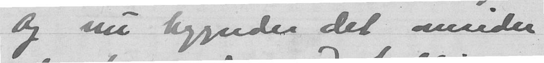Og nu begynder det omsider
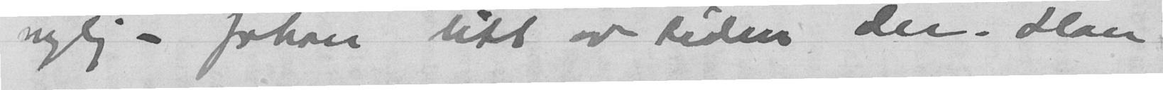nylig - Johan litt av tiden der. Han
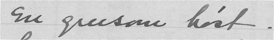En grusom høst.
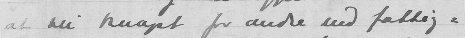at bli knapt for andre end fattig-
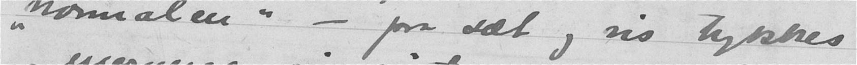"normalen" - paa sæt og vis trykkes
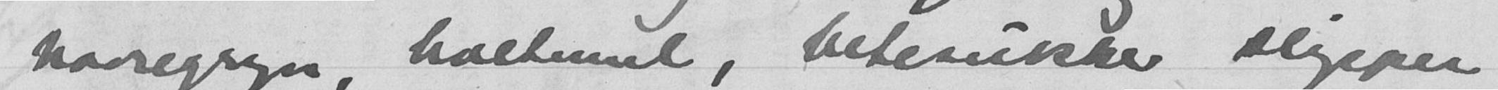havregryn, hvetemel, betesukker slipper
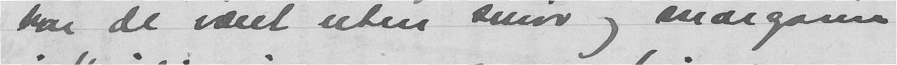har de været uten smør og margarin
